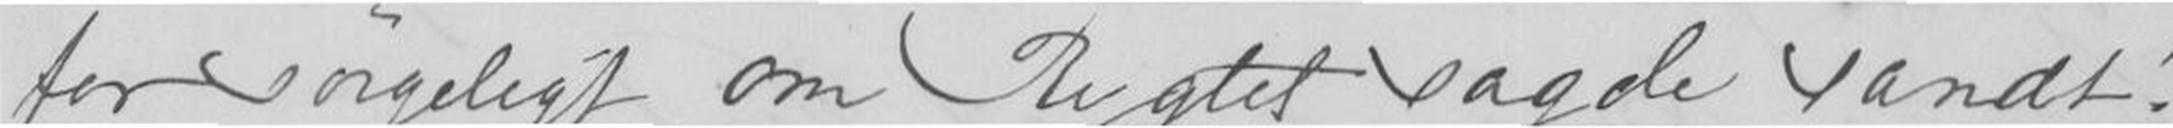for sørgeligt om Rygtet sagde sandt!
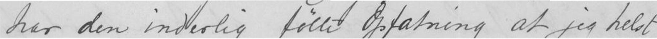har den inderlig følte Opfatning at jeg helst
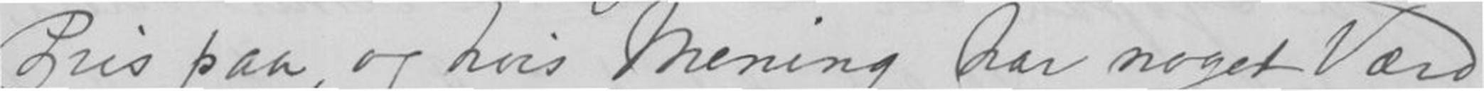Pris paa, og hvis Mening har noget Værd
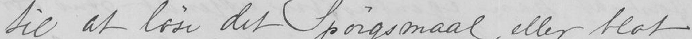til at løse det Spørgsmaal, eller blot
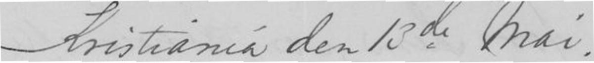Kristiania den 13de Mai
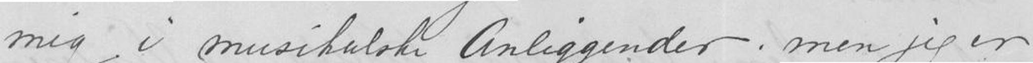mig i Musikalske Anliggender; men jeg er
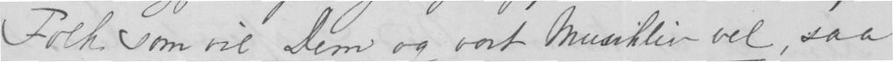Folk som vil Dem og vort Musikliv vel, saa
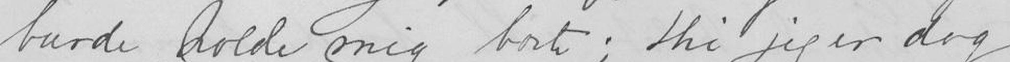burde holde mig borte; thi jeg er dog
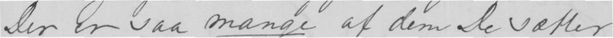Der er saa mange af dem De sætter
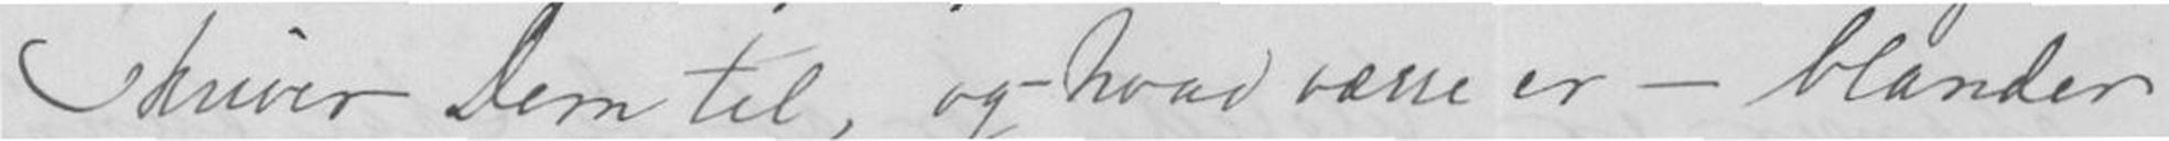skriver Dem til, og-hvad værre er - blander
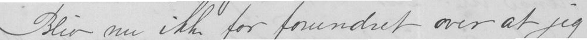Bliv nu ikke for forundret over at jeg
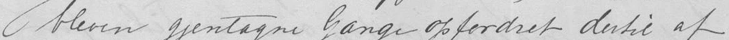bleven gjentagne Gange opfordret dertil af
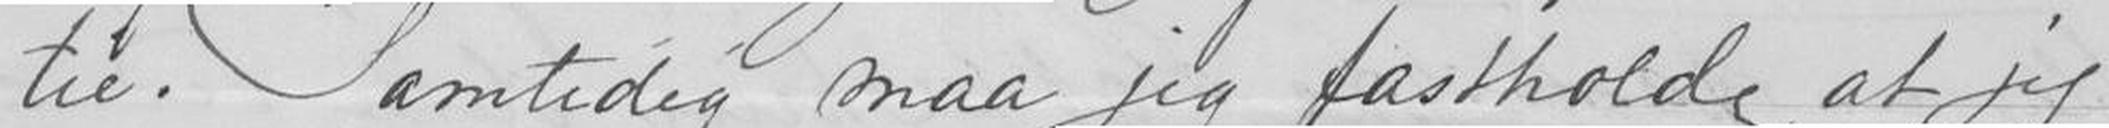tie. Samtidig maa jeg fastholde, at jeg
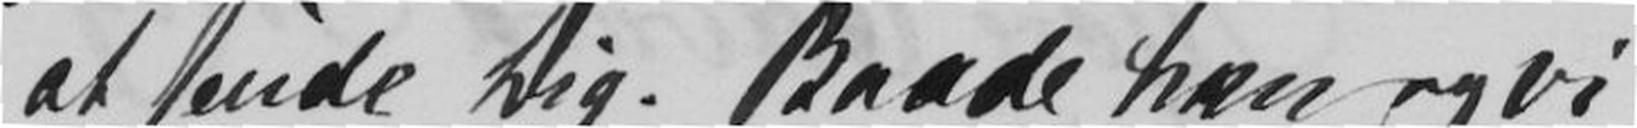at sende Dig. Baade han og vi
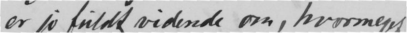er jo fuldt vidende om, hvormeget
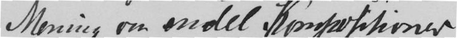Mening om en del Kompositioner,
